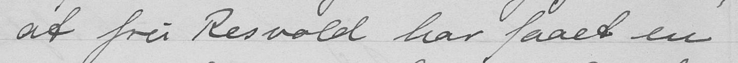at fru Resvold har faaet en
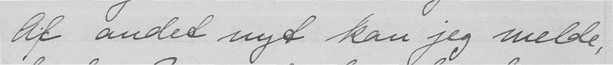Af andet nyt kan jeg melde,
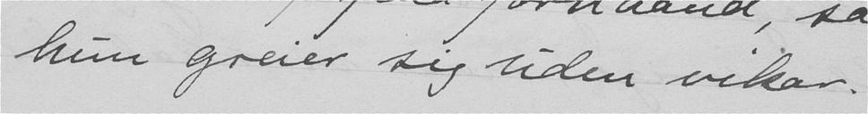hun greier sig uden vikar.
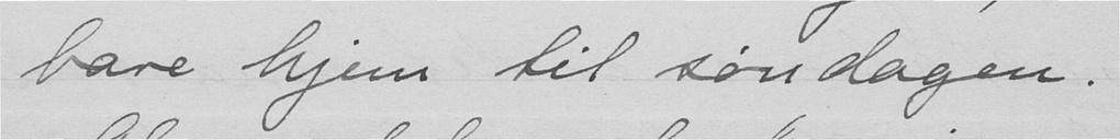bare hjem til søndagen.
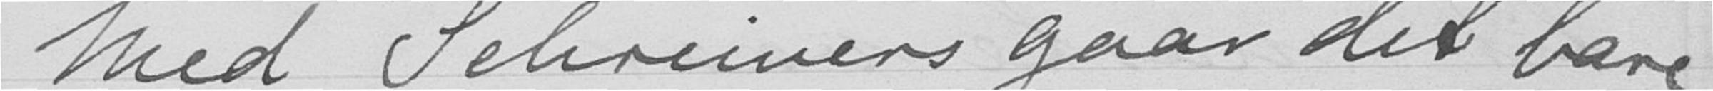Med Schreiners gaar det bare
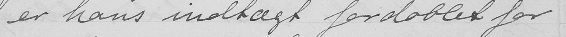er hans indtægt fordoblet for
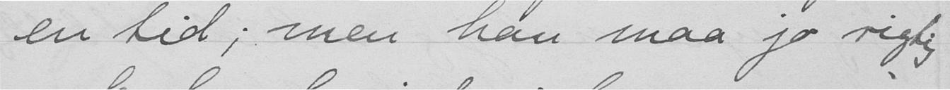en tid; men han maa jo rigtig
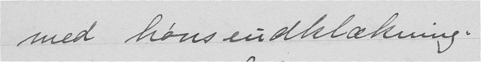med hønseudklækning.
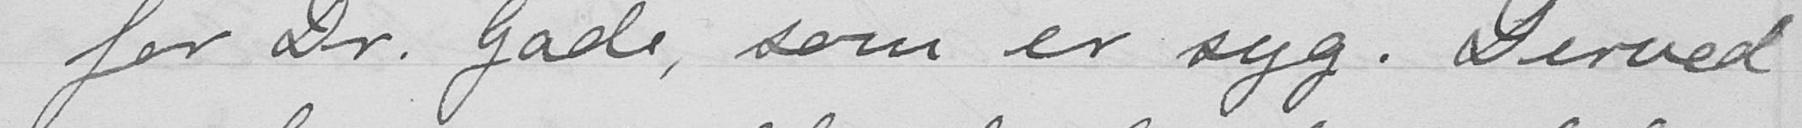for Dr. Gade, som er syg. Derved
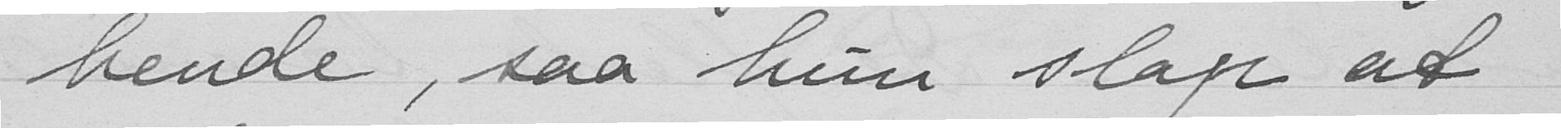hende, saa hun slap at
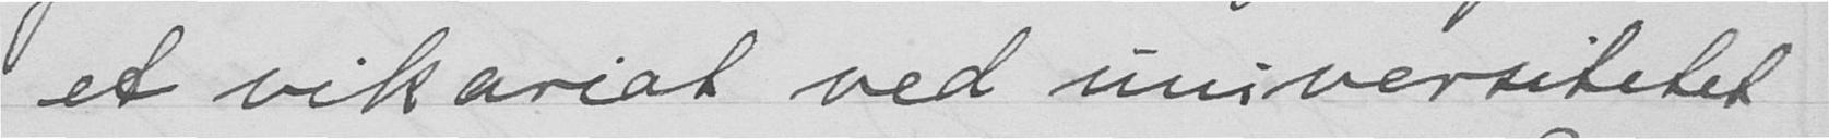et vikariat ved universitetet
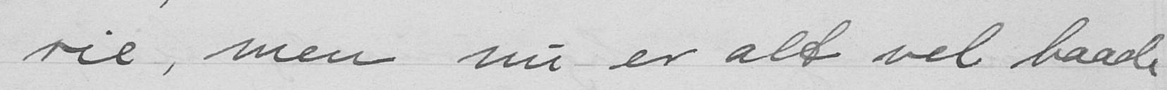rie, men nu er alt vel baade
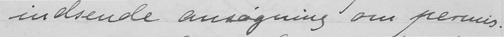indsende ansøgning om permis-
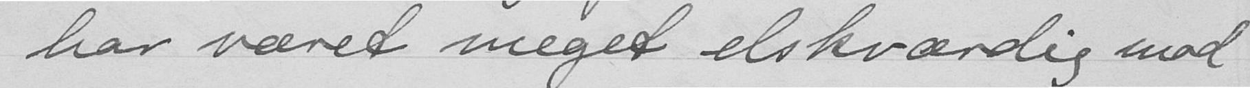har været meget elskværdig mod
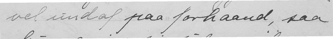vel undaf paa forhaand, saa
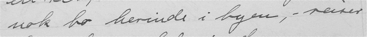nok bo herinde i byen, - reiser
